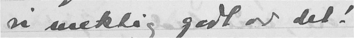vi unektelig godt av det!
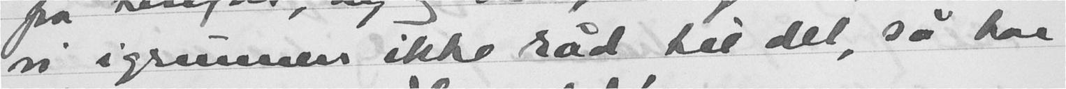vi igrunnen ikke råd til det, så har
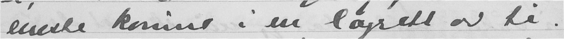eneste kvinne i en lagrett av ti.
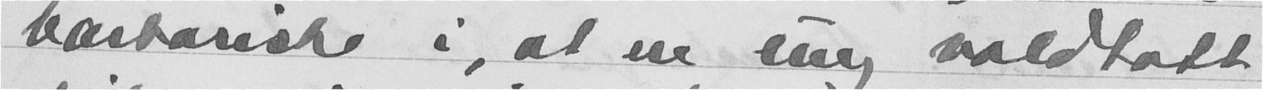barbariske i at en ung voldtatt
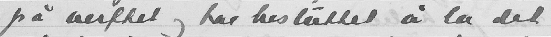på verftet og har besluttet å la det
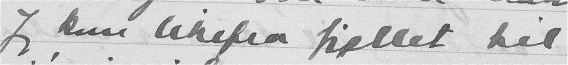Jeg kom likefra fjellet til
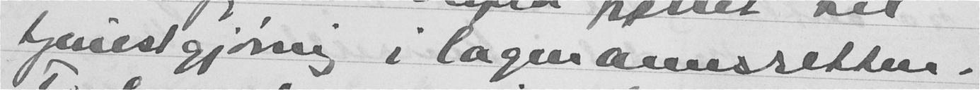tjenestgjørnig i lagmannsretten.
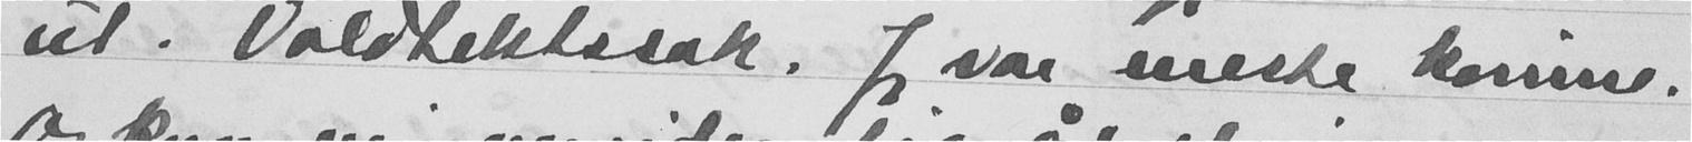ut. Voldtektssak, Jeg var eneste kvinne.
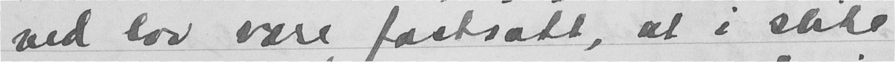ved lov være fastsatt, at i slike
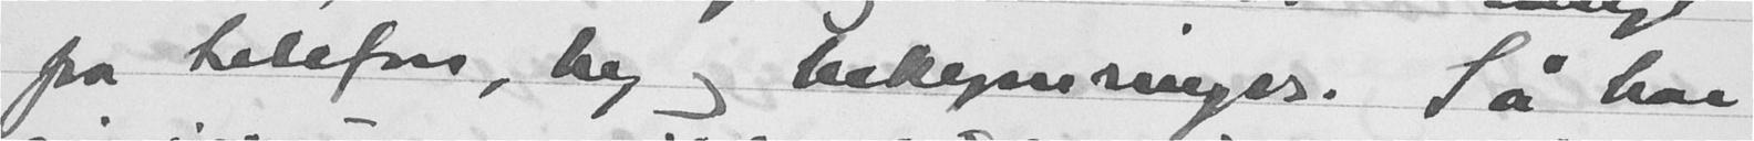fra telefon, by og bekymringer. Så har
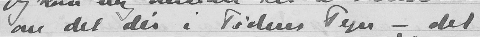om det der i Tidens Tegn - det
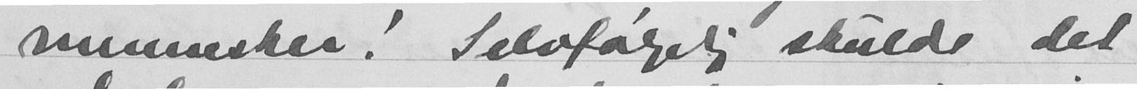Mennesker! Selvfølgelig skulde det
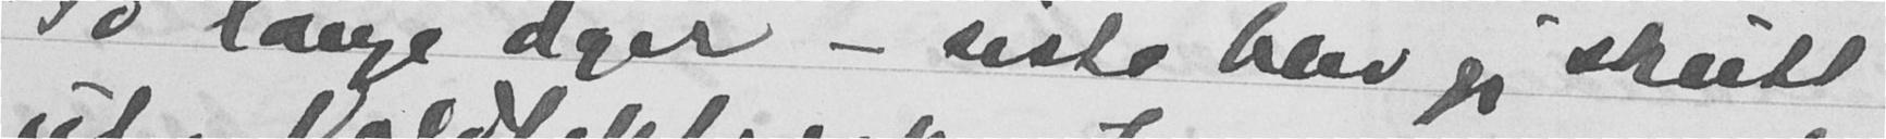To lange dager - siste blev jeg skutt
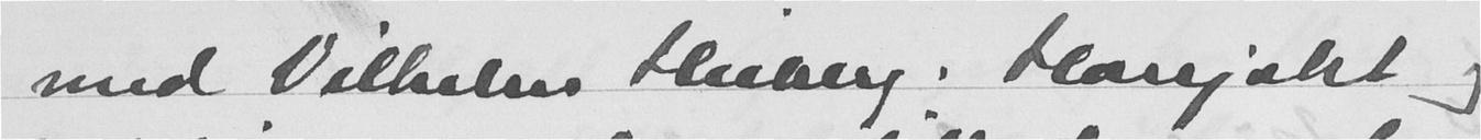med Vilhelm Heiberg. Harejakt og
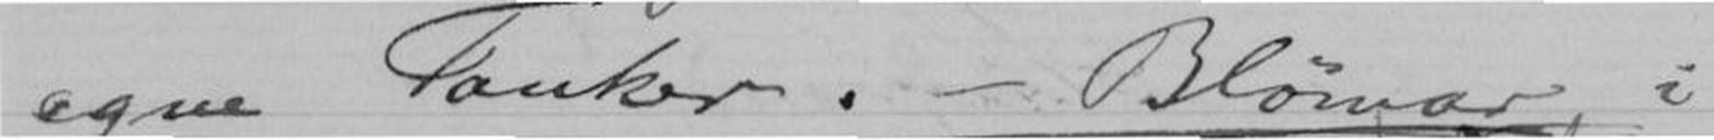egne Tanker. - Blømar i
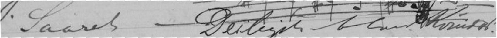Saaret Deiligst blandt Kvinder!!
